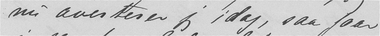nu averterer jeg idag, saa faar
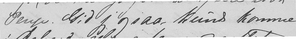Penge. Gid jeg ogsaa kunde komme
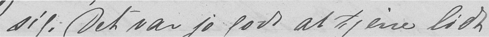sig. Det var jo godt at tjene lidt
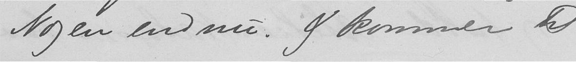Nogen endnu. Jeg kommer til
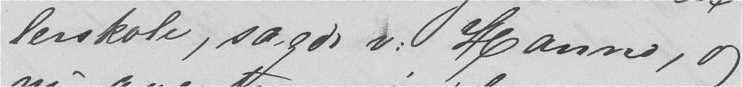lerskole, sagde v: Hanno, og
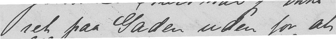ret paa Gaden uden for at
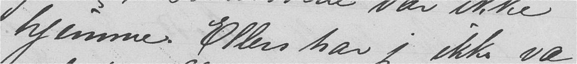hjemme. Ellers har jeg ikke væ-
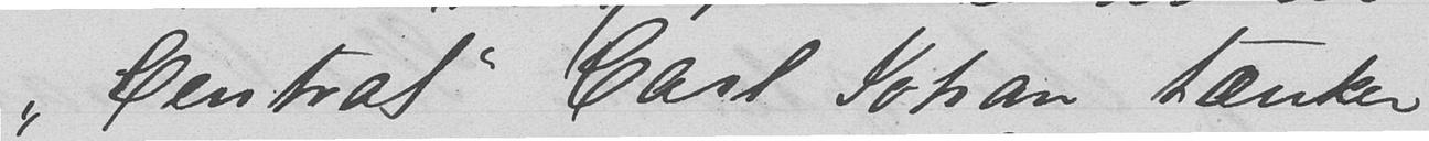"Central" Carl Johan tænker
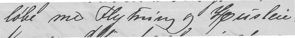løbe med Flytning og Husleie.
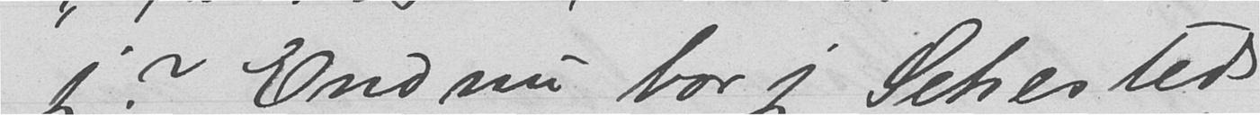jeg? Endnu bor jeg Sehesteds
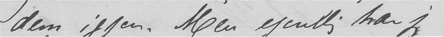dem igjen. Men egentlig har jeg
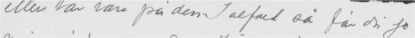eller tar vare pa den. Ialfald, så får du jo
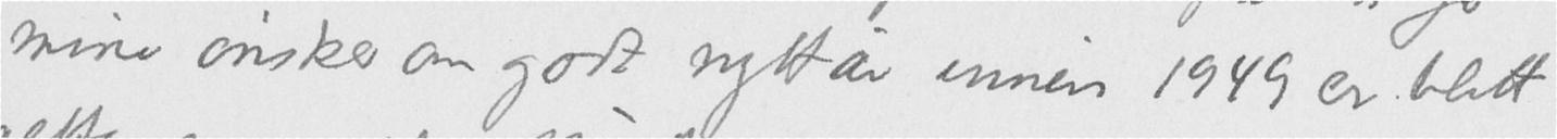mine ønsker om godt nyttår innen 1949 er blitt
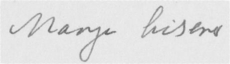Mange hilsener
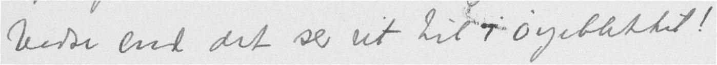bedre end det ser ut til i øyeblikket!
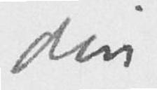din
